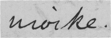mørke.
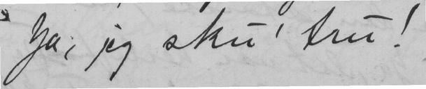Ja, jeg sku' tru!
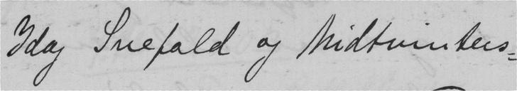Idag Snefald og Midtviters-
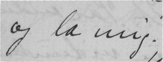og la mig.
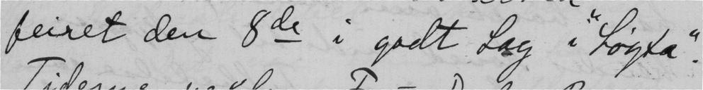feiret den 8de i godt Lag i "Løgta".
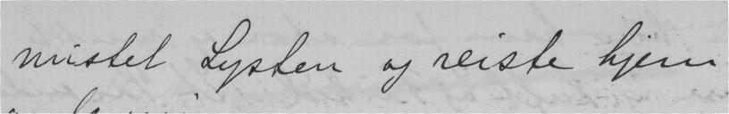mistet Lysten og reiste hjem
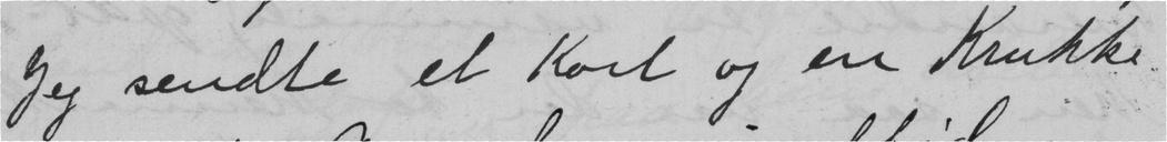Jeg sendte et Kort og en Krukke.
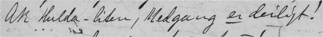Ak Hulda - liten, Medgang er deiligt!
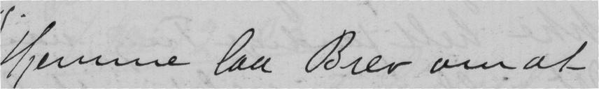Hjemme laa Brev om at
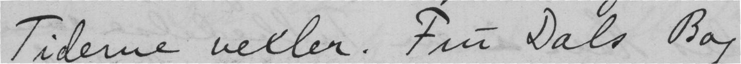Tiderne veller. Fru Dals Bog
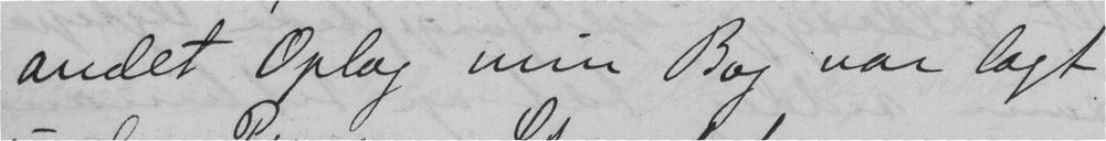andet Oplag min Bog var lagt
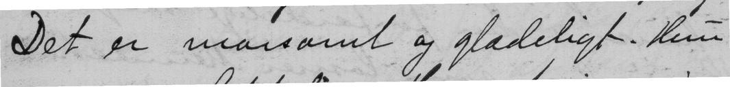Det er morsomt og gladeligt. Hun
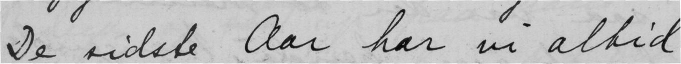De sidste aar har vi altid
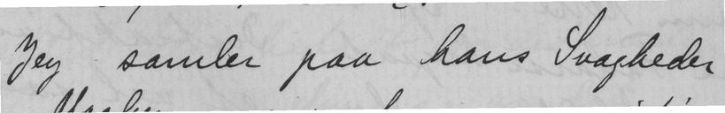Jeg samler paa hans Svagheder
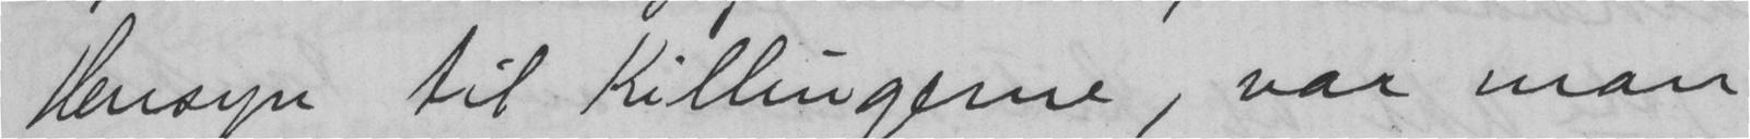Hensyn til Killingerne, var man
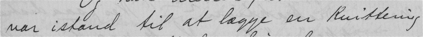var istand til at lægge en Kvittering
